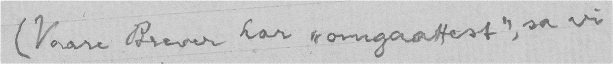(Vaare Brever har "omgaattest", sa vi
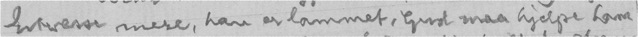lnteresse mere, han er lammet, Gud maa hjelpe ham
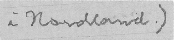i Nordland.)
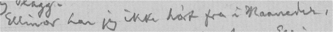Ellinor har jeg ikke hørt fra i Maaneder,
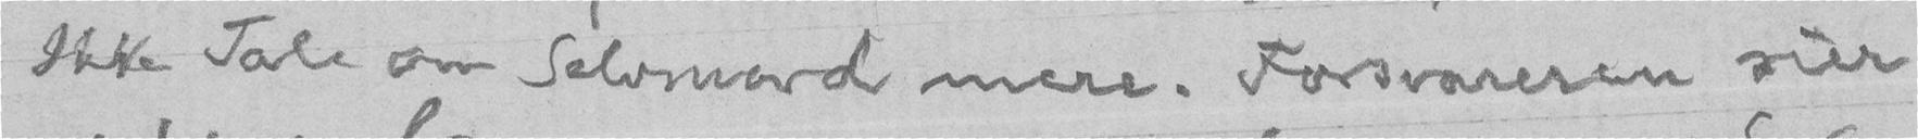Ikke Tale om Selvmord mere. Forsvareren sier
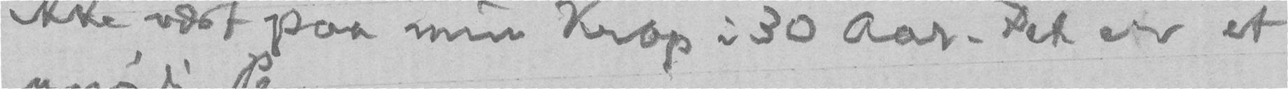ikke vært paa min Krop i 30 Aar. Det er et
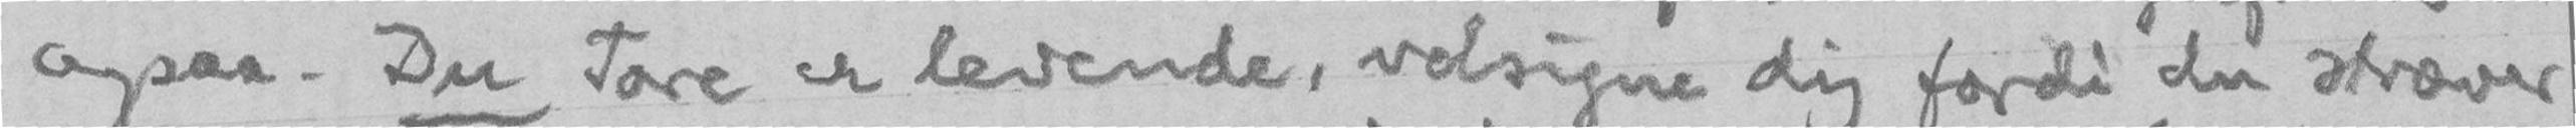ogsaa. Du Tore er levende, velsigne dig fordi du stræver
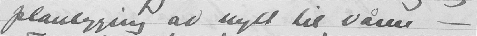planligging av nytt til våren -
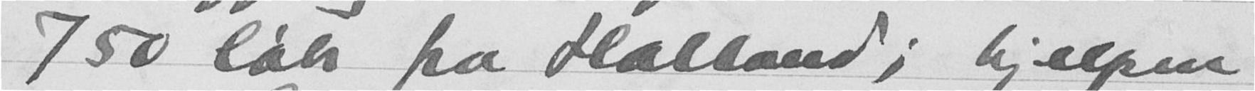750 løk fra Holland; hjelpen
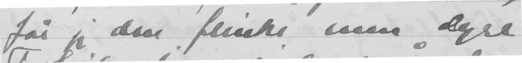får jeg den flinke men dyre
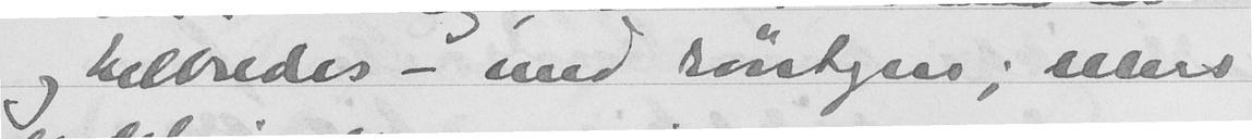og helbredes - med røntgen; ellers
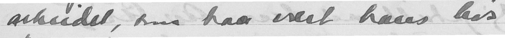arbeidet, som har vært, hans livs
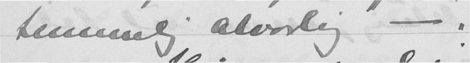temmelig alvorlig -.
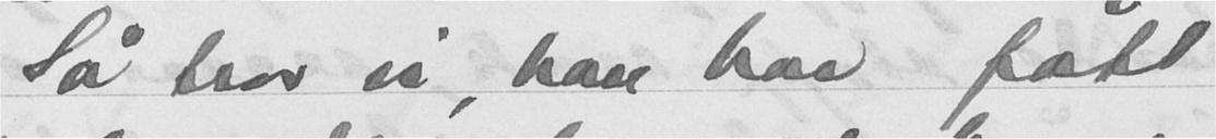Så tror vi, han har fått
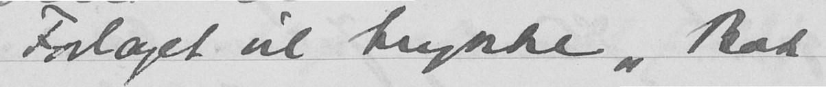Forlaget vil trykke "Bok
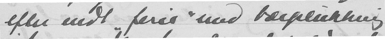efter endt "Ferie" med bærplukking
